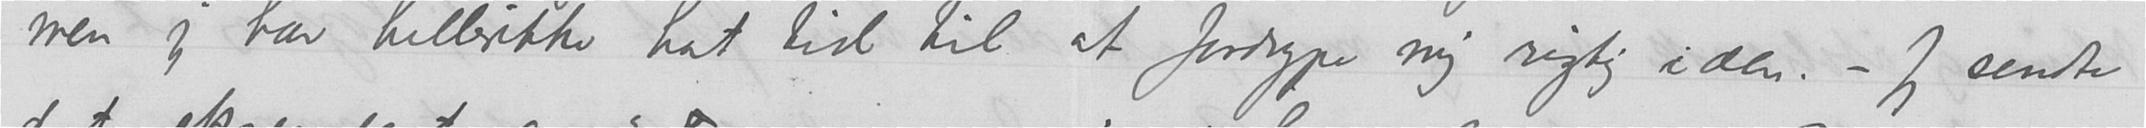men jeg har hellerikke hat tid til at fordype mig rigtig i den. – Jeg sendte
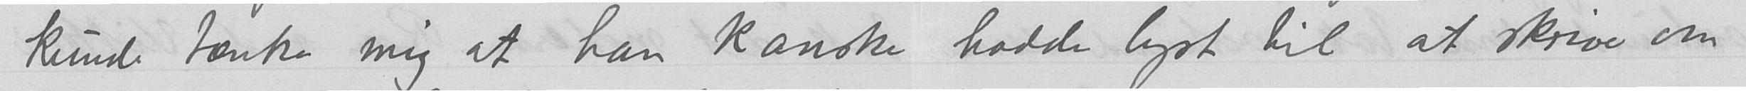kunde tænke mig at han kanske hadde lyst til at skrive om
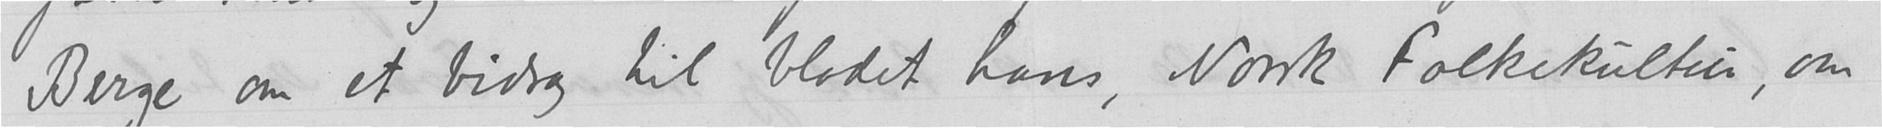Berge om et bidrag til bladet hans, Norsk Folkekultur, om
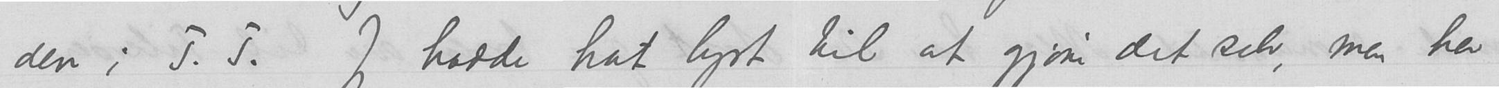den i T.T. Jeg hadde hat lyst til at gjøre det selv, men her
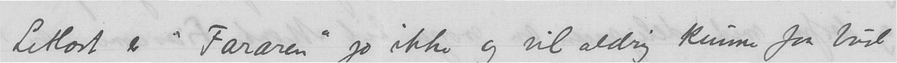Letlæst er "Fararen" jo ikke og vil aldrig kunne faa bud
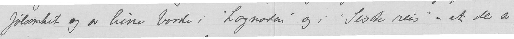følsomhet og av lune baade i "Lagnaden" og i "Sisste reis" – at der er
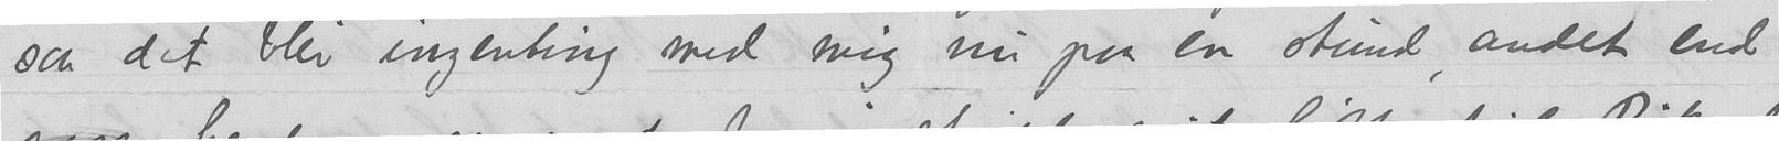saa det blir ingenting med mig nu paa en stund, andet end
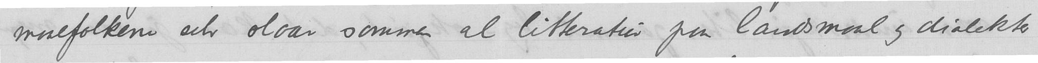maalfolkene selv slaar sammen al litteratur paa landsmaal og dialekter
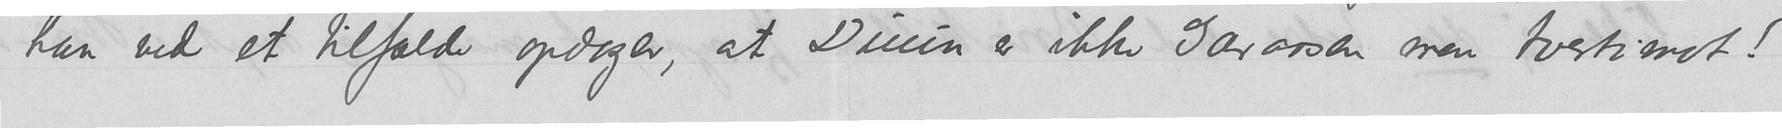han ved et tilfælde opdager, at Duun er ikke Garaasen, men tvertimot!
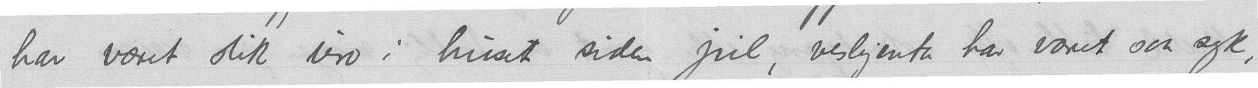har været slik uro i huset siden jul, veslejenta har været saa syk,
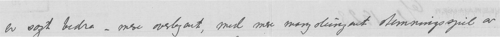er sagt bedre – mere overlegent, med mere mangslungent stemningsspil av
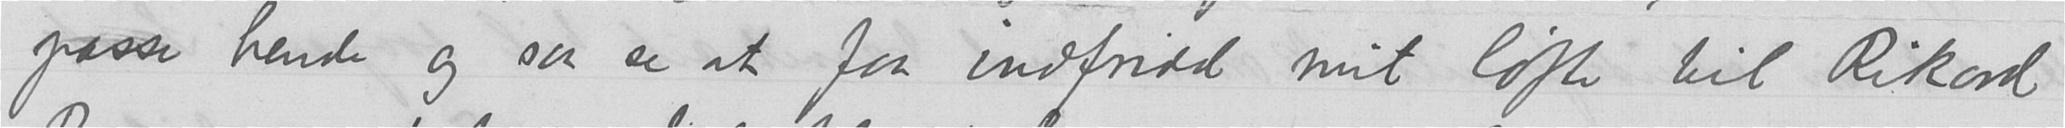passe hende og saa se at faa indfridd mit løfte til Rikard
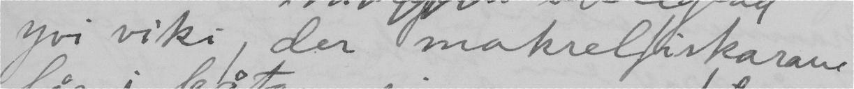yvi viki, der makrelfiskarane
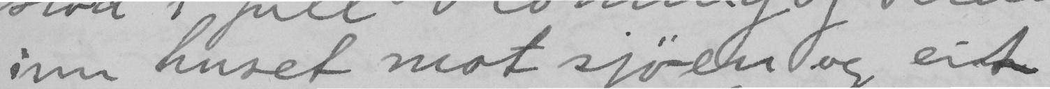inn huset mot sjöen og eit
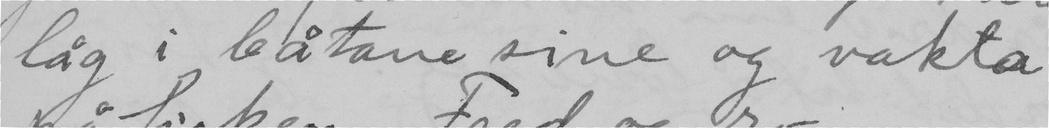låg i båtane sine og vakta
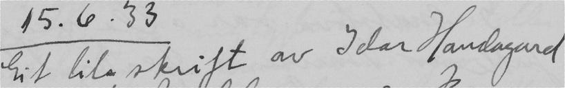Eit lite skrift av Idar Handagard
